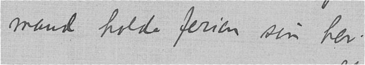mand holde ferien sin her.
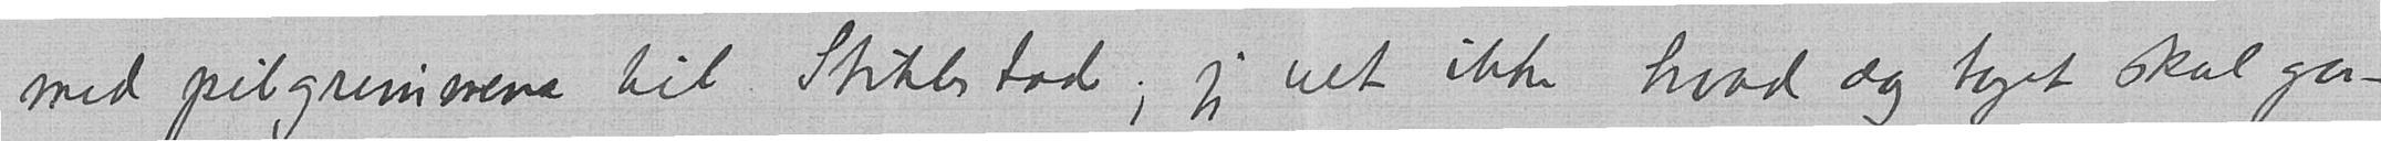med pilgrimmene til Stiklestad; jeg vet ikke hvad dag toget skal gaa –
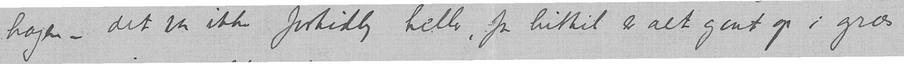hagen – det var ikke fortidlig heller, for hittil er alt gaat op i græs
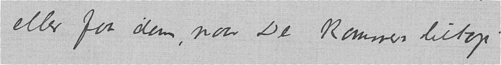eller faa dem, naar De kommer hitop.
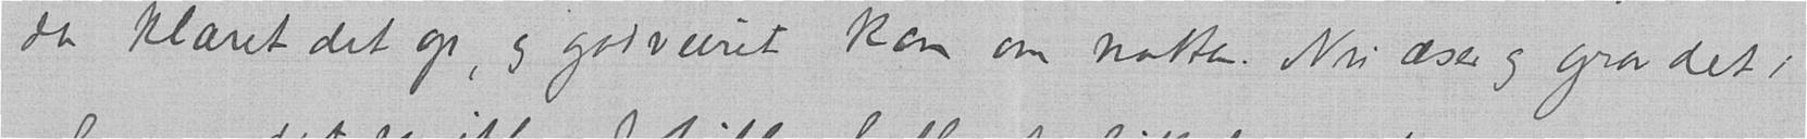da klaret det op, og godveiret kom om natten. Nu æser og gror det i
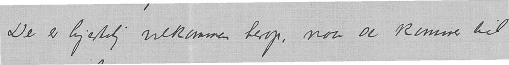De er hjertelig velkommen herop, naar De kommer til
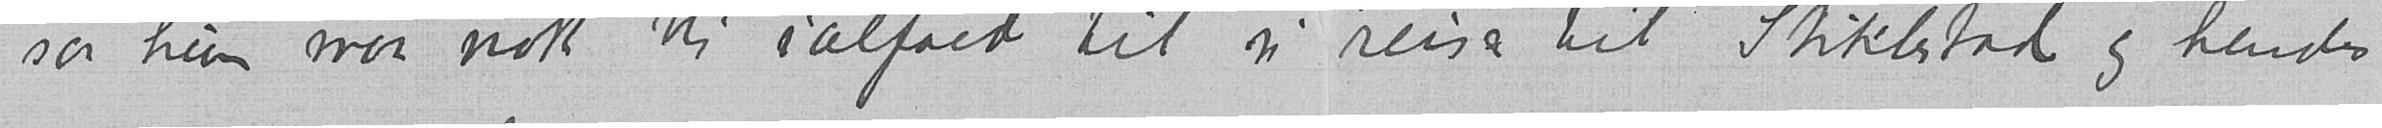saa hun maa nok bli ialfald til vi reiser til Stiklestad og hendes
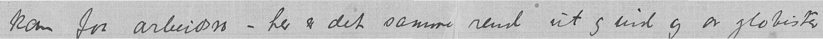kan faa arbeidsro – her er det samme rend ut og ind og av globister
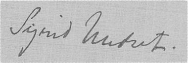Sigrid Undset.
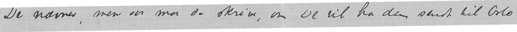De nævner, men saa maa de skrive, om De vil ha dem sendt til Oslo
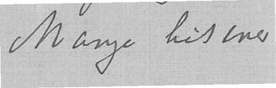Mange hilsener
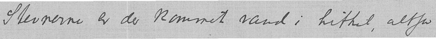Stevnerne er der kommet vand i hittil, altfor
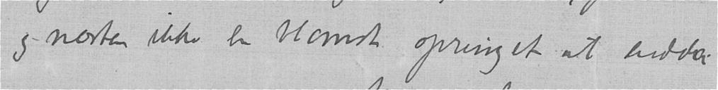og næsten ikke en blomst sprunget ut endda.
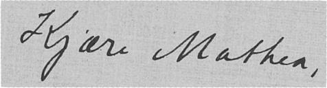Kjære Mathea,
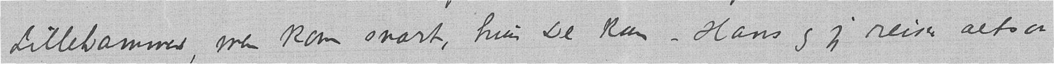Lillehammer, men kom snart, hvis De kan – Hans og jeg reiser altsaa
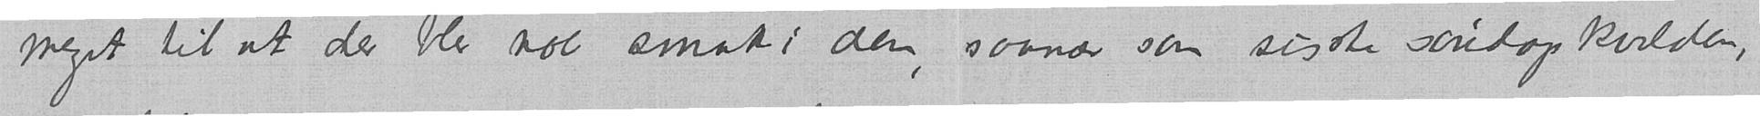meget til at der blev noe smak i dem, saanær som sisste søndagskvelden,
